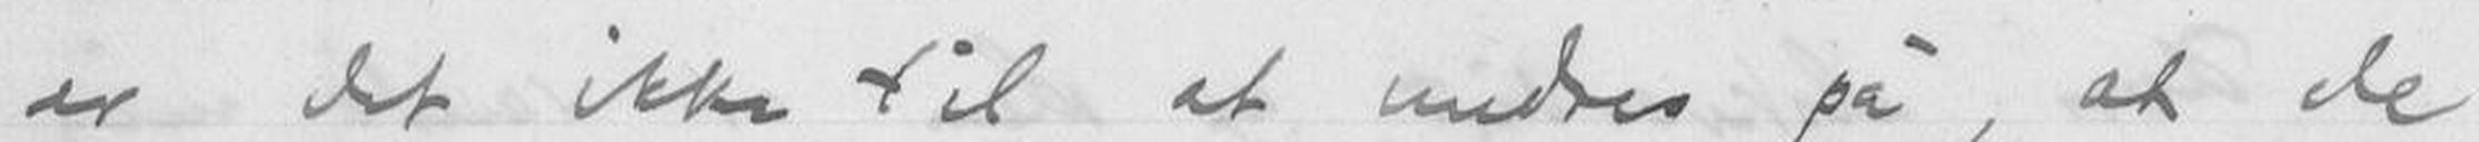er det ikke til at undres på, at de
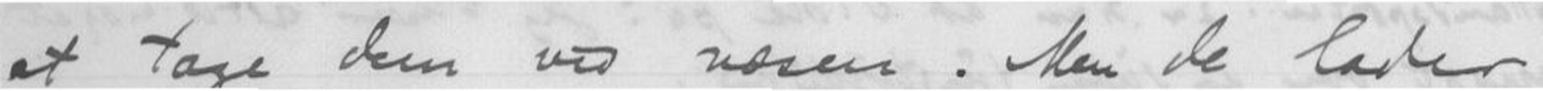at tage dem ved næsen. Men de lader
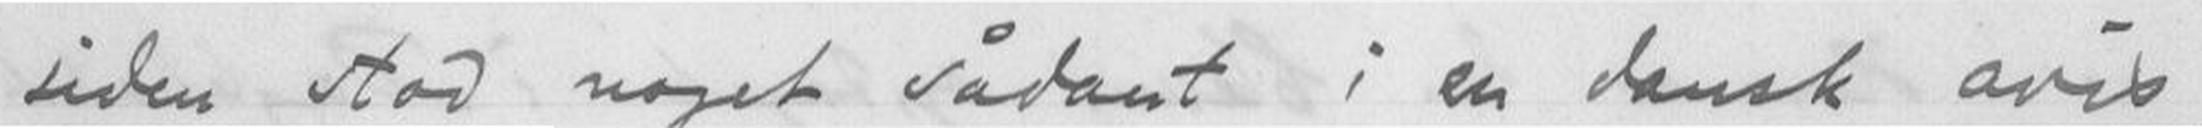siden stod noget sådant i en dansk avis,
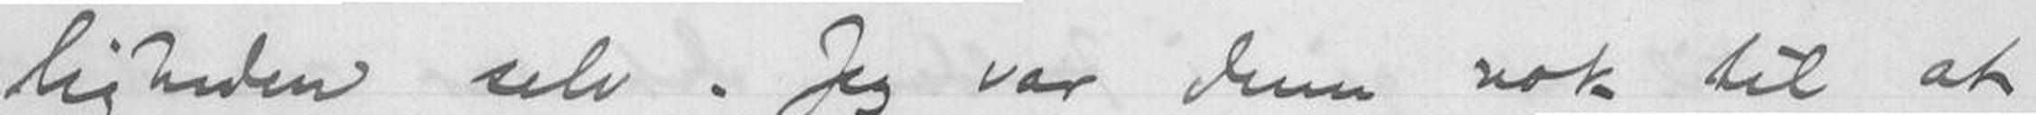ligheden selv. Jeg var dum nok til at
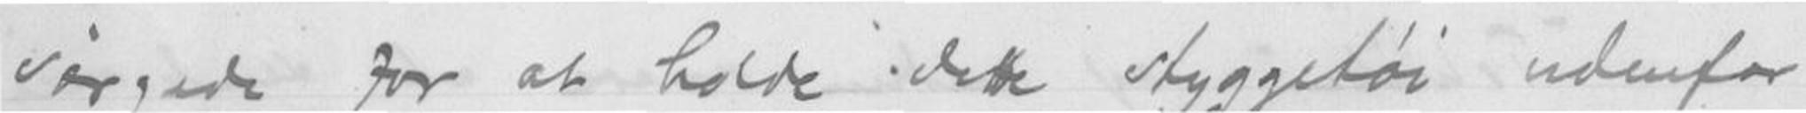sørgede for at holde dette styggetøi udenfor
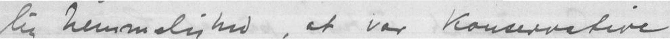lig hemmelighed, at vor konservative
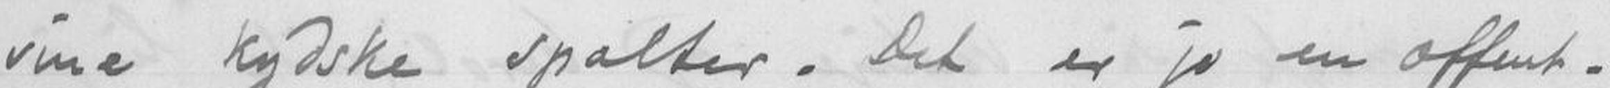sine kydske spalter. Det er jo en offent-
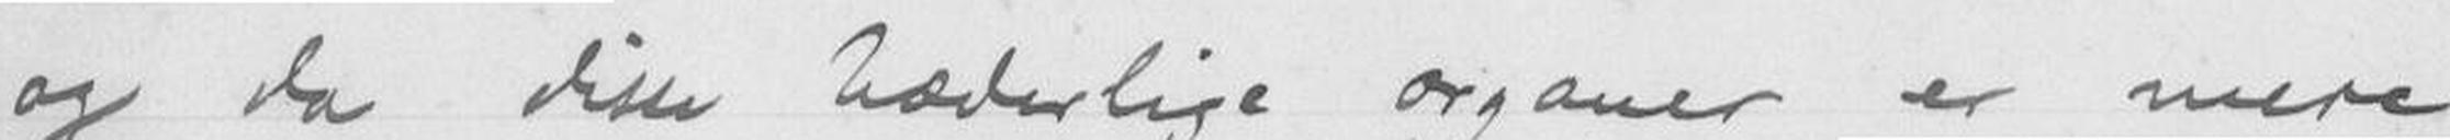og da disse hæderlige organer er mere
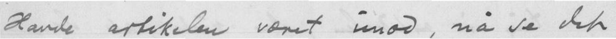Havde artikelen været imod, nå se det
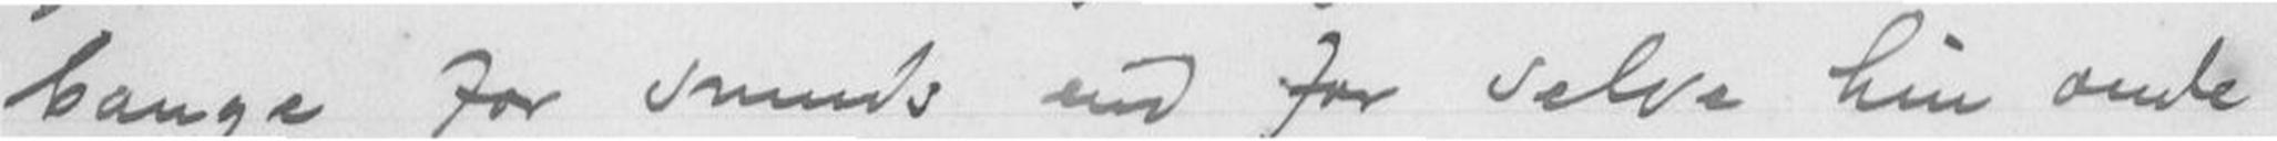bange for smuds end for selve hin onde
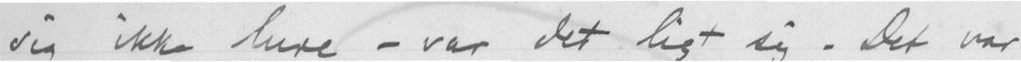sig ikke lure - var det ligt sig. Det var
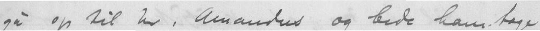gå op til hr. Amandus og bede ham tage
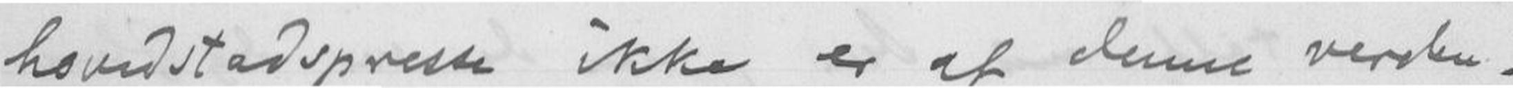hovedstadspresse ikke er af denne verden.
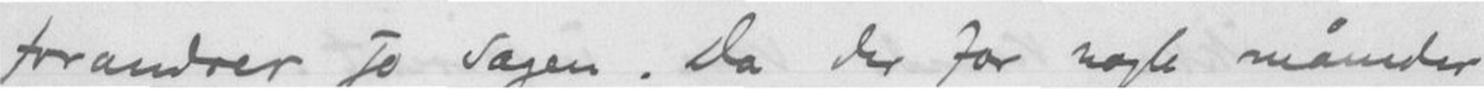forandrer jo sagen. Da der for nogle måneder
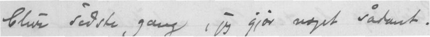blive sidste gang, jeg gjør noget sådant.
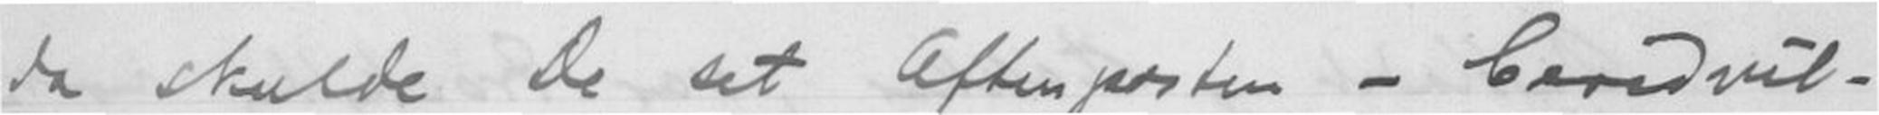da skulde De set Aftenposten - berædvil-
